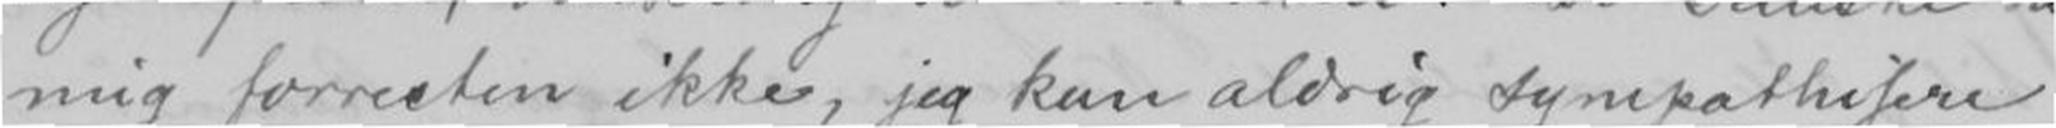mig forresten ikke, jeg kan aldrig sympathisere
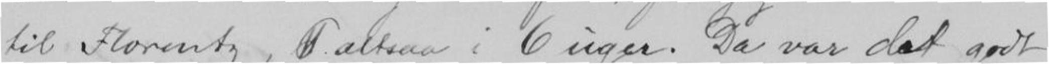til Florentz, altsaa i 6 uger. Da var det godt
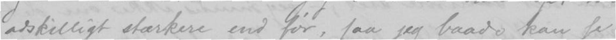adskilligt stærkere end før, saa jeg baade kan se
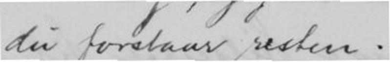du forstaar resten.
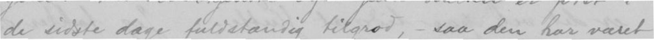de sidste dage fulstendig tilgrod, saa den har været
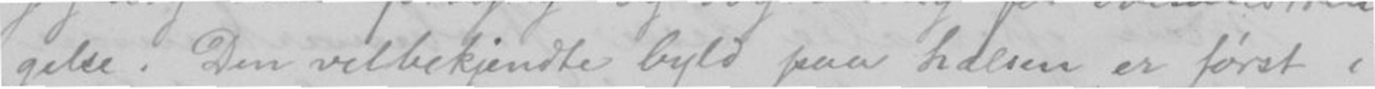gelse. Den velbekjendte byld paa halsen er først i
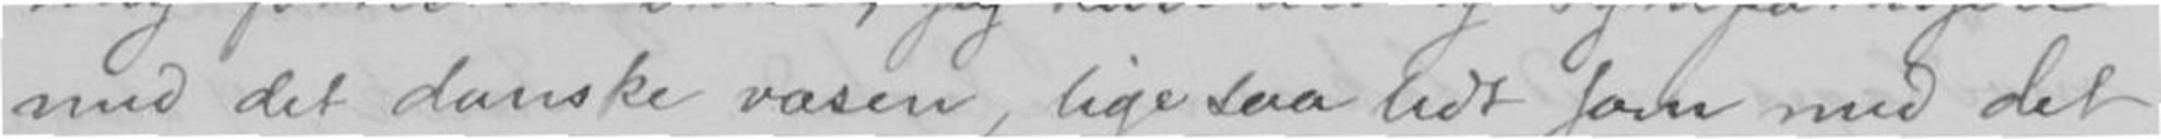med det danske væsen, ligesaa lidt som det
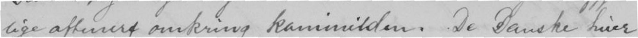lige aftener omkring kaminilden. De Danske huer
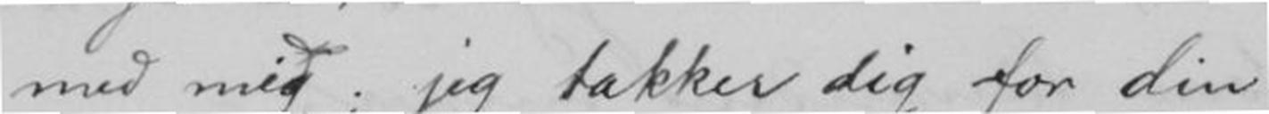med mig; jeg takker dig for din
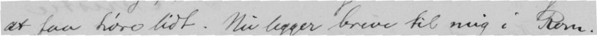at faa høre lidt. Nu ligger breve til mig i Rom.
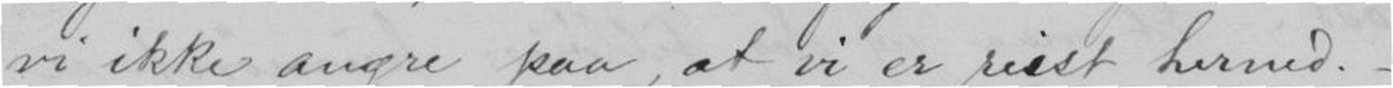vi ikke angre paa, at vi er reist herned. -
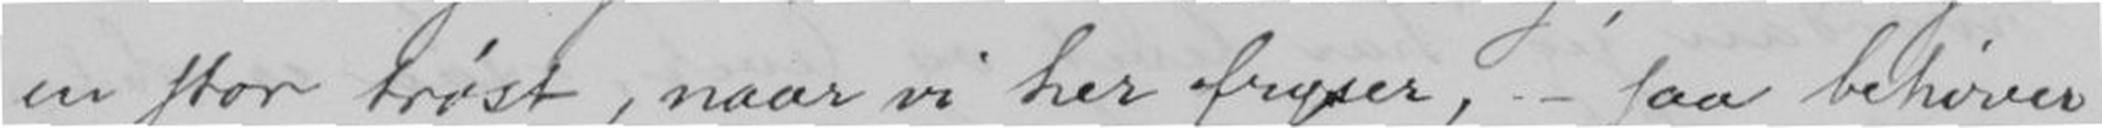en stor trøst, naar vi her fryser, - saa behøver
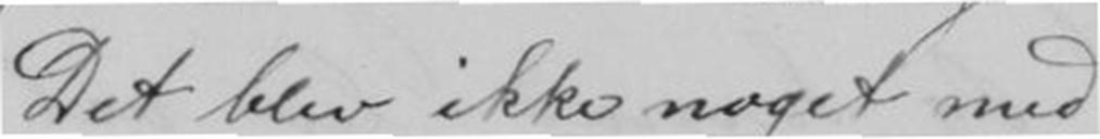Det blev ikke noget med
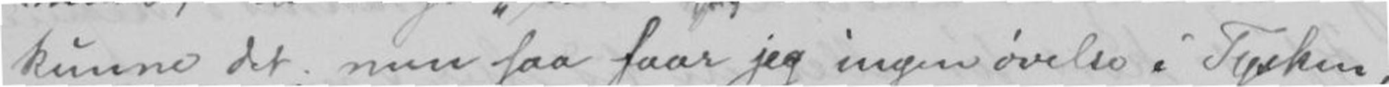kunne det; men saa faar jeg ingen øvelse i Tysken,
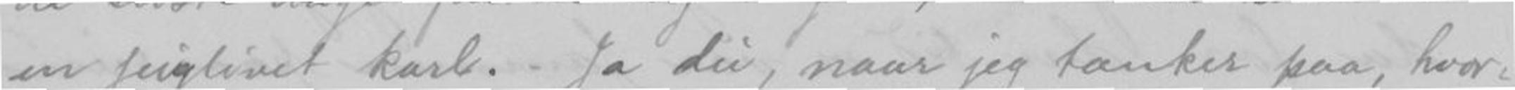en seiglivet karl. - Ja du, naar jeg tænker paa, hvor-
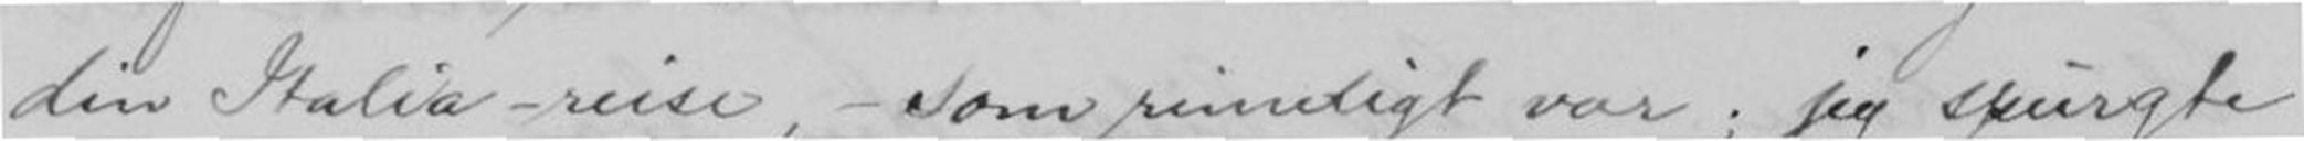din Italia-reise, - som rimeligt var; jeg spurgte
